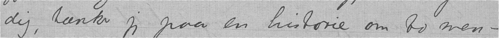dig, tænker jeg paa en historie om to men-
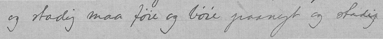og stadig maa føie og bøie paanyt og stadig
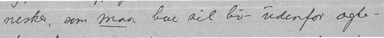nesker, som maa leve sitt liv udenfor ægte-
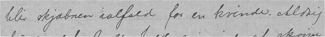blev skjæbnen ialfald for en kvinde. Aldrig
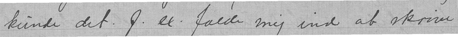kunde det f.ex. falde mig ind at skrive
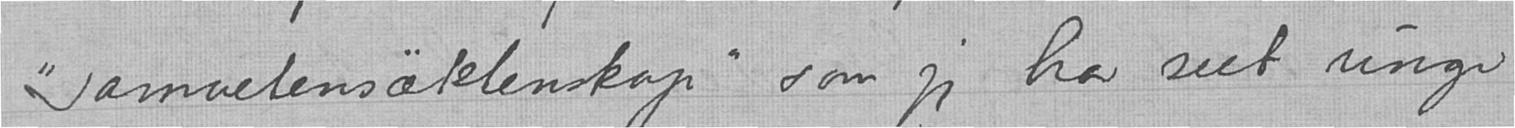"samvetensäkteskap" som jeg har seet unge
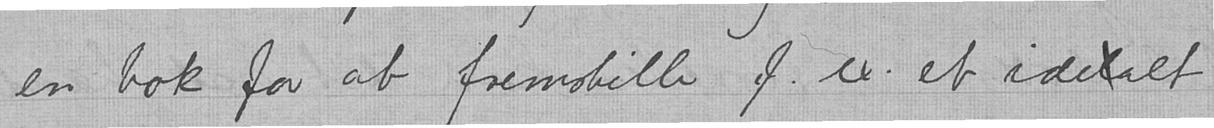en bok for at fremstille f.ex et idelalt
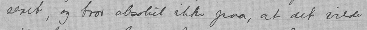seret, og tror absolut ikke paa, at det vilde
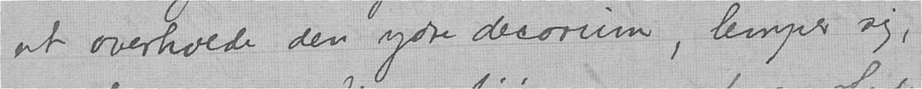at overholde den ydre decorium, lemper sig,
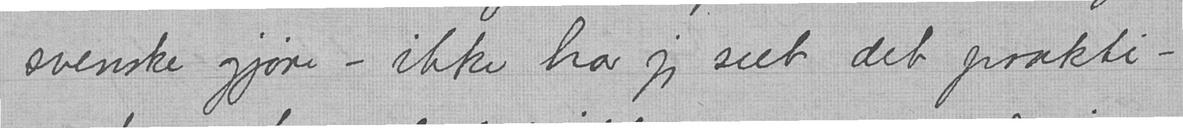svenske gjøre - ikke har jeg seet det prakti-
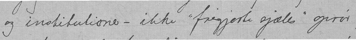og institutione - ikke "frigjorte sjæle" oprør,
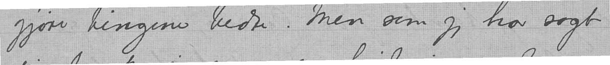gjøre tingene bedre. Men som jeg har sagt
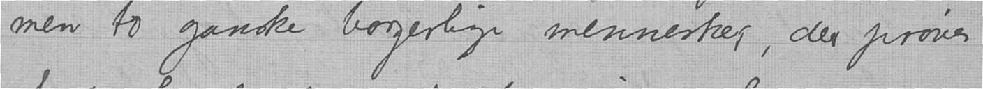men to ganske borgerlige mennesker, der prøver
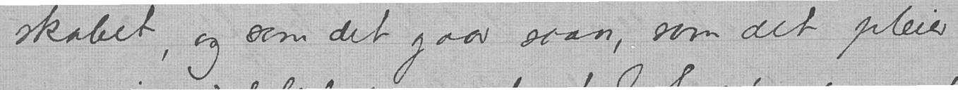skabet, og som det gaar saan, som det pleier
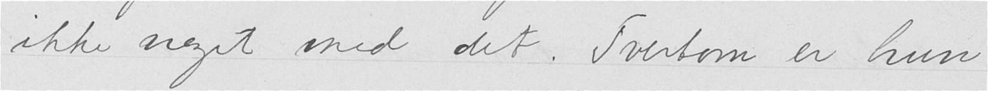ikke noget med det. Tvertom er hun
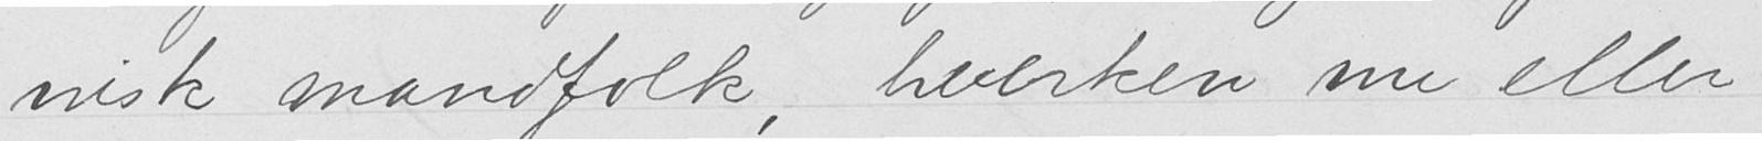nisk mandfolk, hverken nu eller
